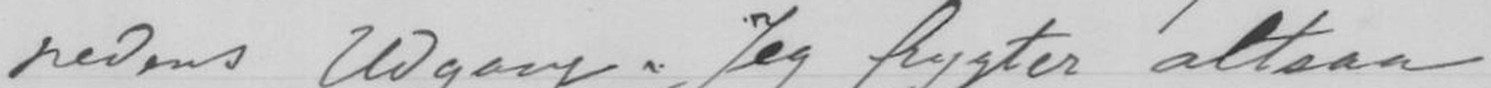nedens Udgang. Jeg frygter altsaa
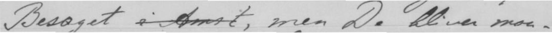Besøget i Amst, men De bliver nor-
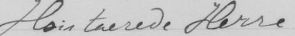Høistaerede Herre
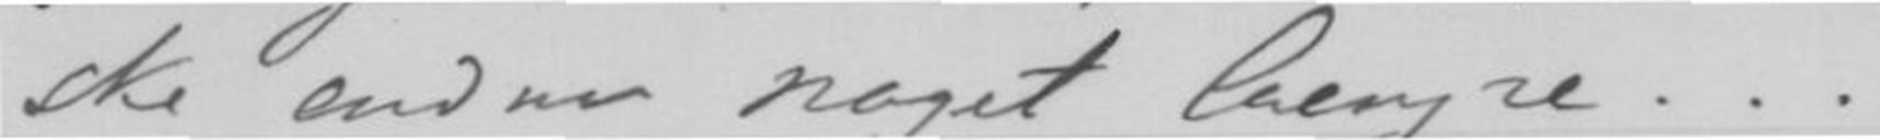ske endnu noget længre.
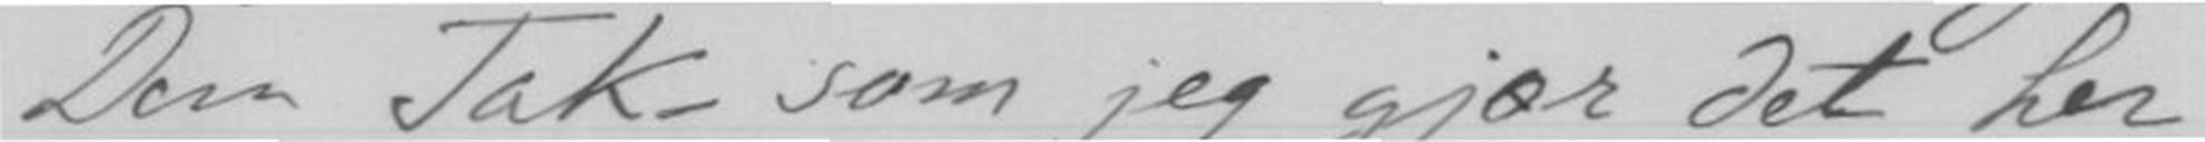Dem Tak som jeg gjør det her
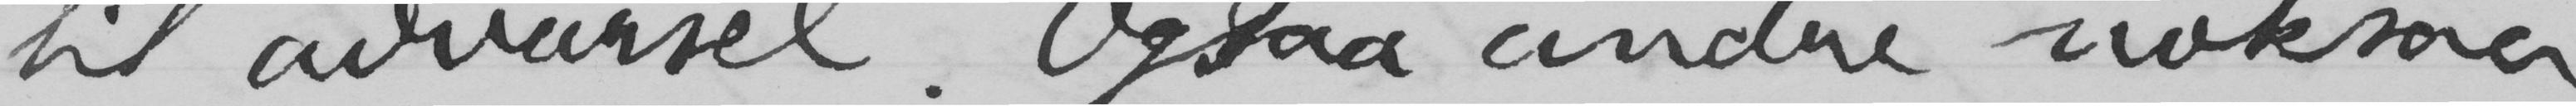til advarsel. Ogsaa andre noksaa
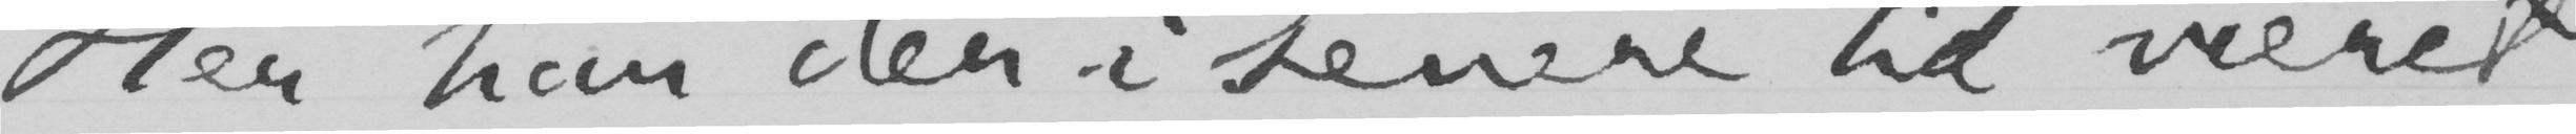Her har der i senere tid været
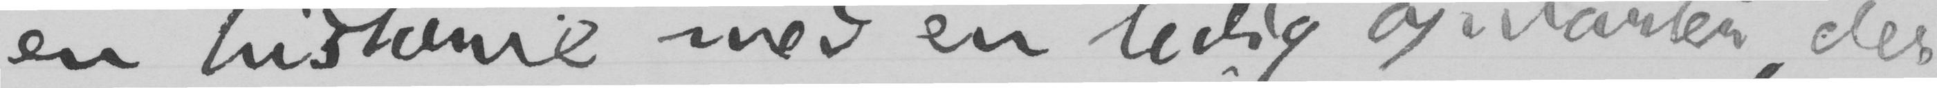en historie med en ledig opvarter, der
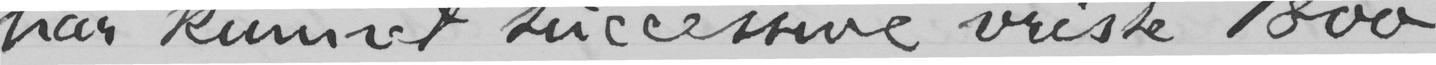har kunnet suvvessive vriste 1800
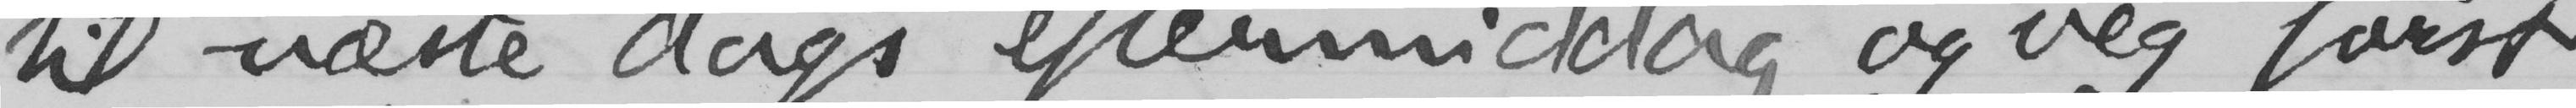til næste dags eftermiddag og veg først,
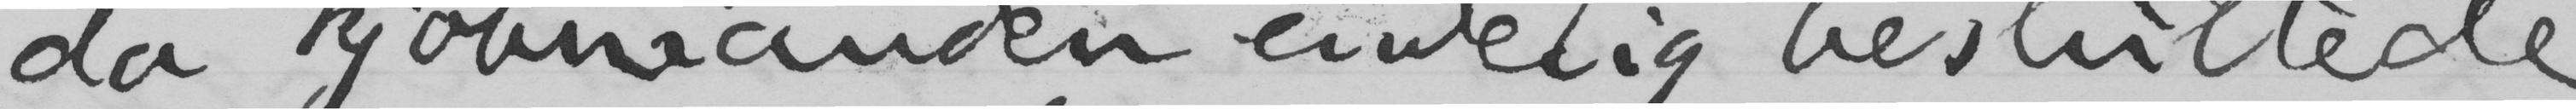da kjøbmanden endelig besluttede
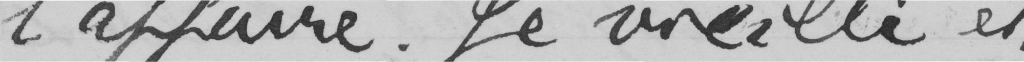l’affaire. Je vicilli et
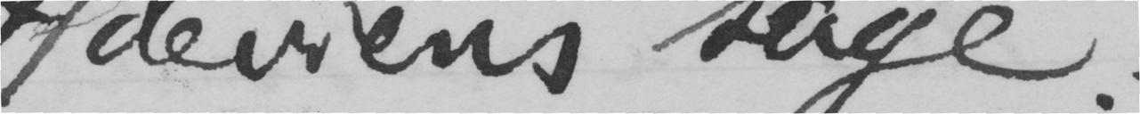deivens sage:
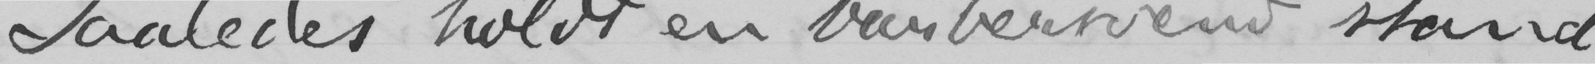Saaledes holdt en barbersvend stand
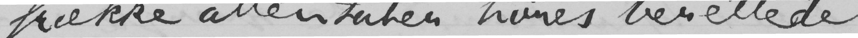frække attentater høres berettede.
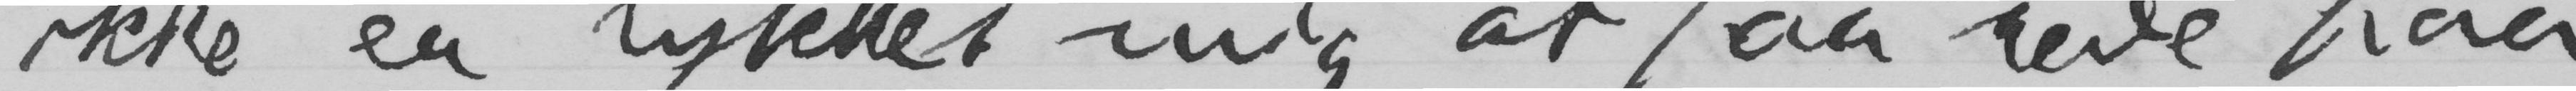ikke er lykkes mig at faa rede paa,
